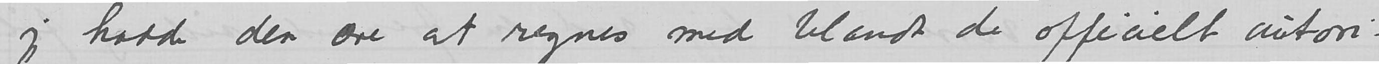jeg hadde den ære at regnes med blant de officielt autori
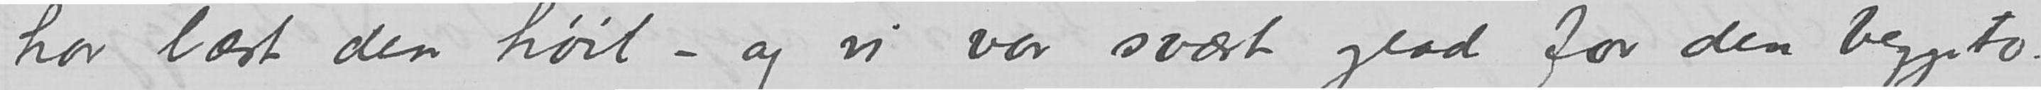har læst den høit - og vi var svært glad for den begge to.
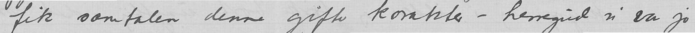fik samtalen denne gifte karakter - herregud vi var jo
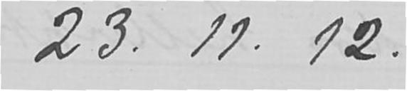23.11.12
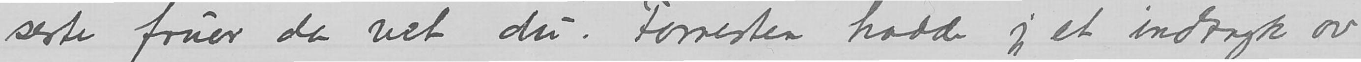serte fruer da vet du. Forresten hadde jeg et indtryk av
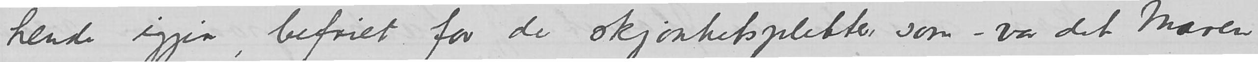se hende igjen, befriet for de skjønhetspletter som - var det Maren
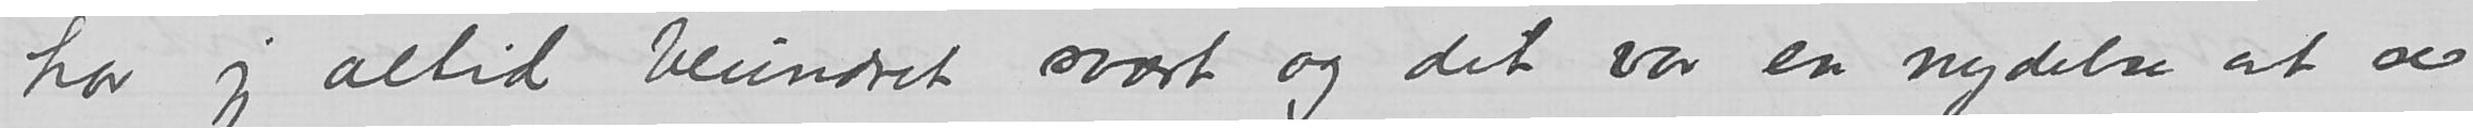har jeg altid beundret svært og det var en nydelse at
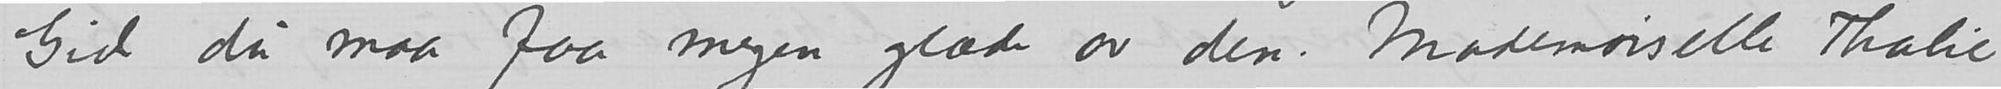Gid du maa faa megen glæde av den. Mademoiselle Thalie
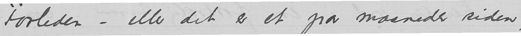Forleden - eller det er et par maaneder siden,
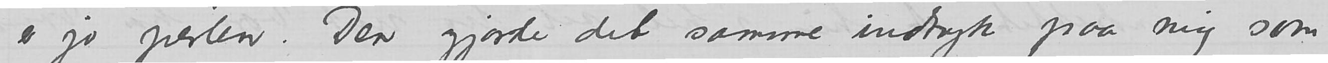er jo perlen. Den gjorde det samme indtryk paa mig som
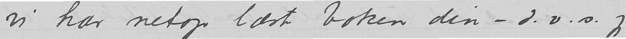vi har netop læst boken din - d.v.s. jeg
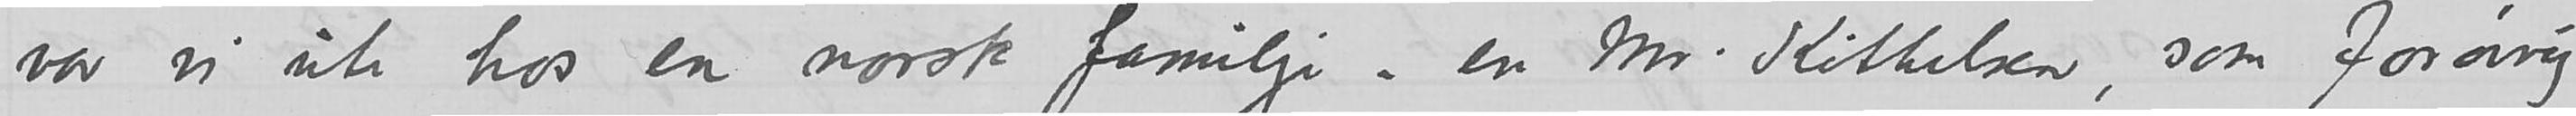var vi ute hos en norsk familje - en Mr. Kittelsen, som forøvrig
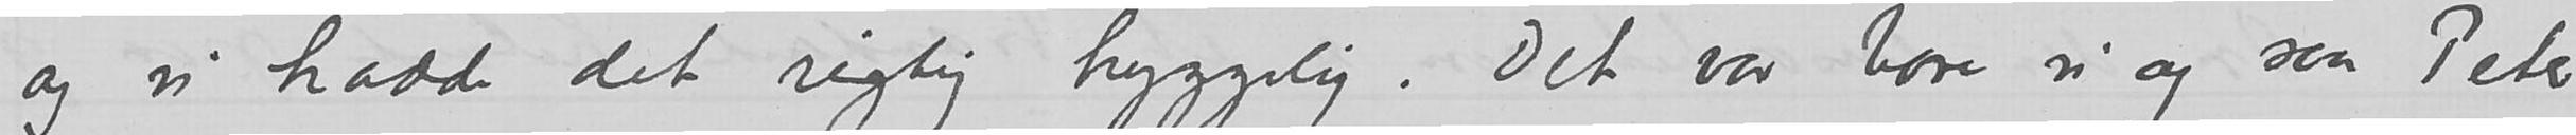og vi hadde det rigtig hyggelig. Det var bare vi og saa Peter
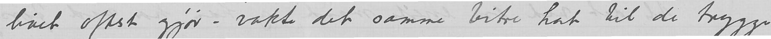livet oftest gjør – vakte det samme bitre hat til de trygge
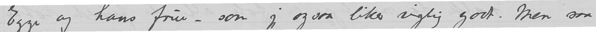Egge og hans frue - som jeg ogsaa liker rigtig godt. Men saa
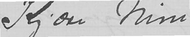Kjære Nini
